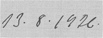13.8.1926.
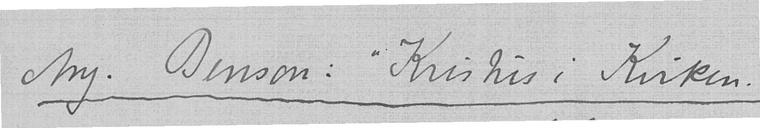Ang. Benson: "Kristus i Kirken."
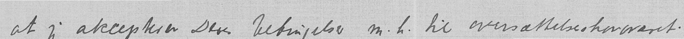at jeg akcepterer Deres betingelser m.h. til oversættelseshonoraret.
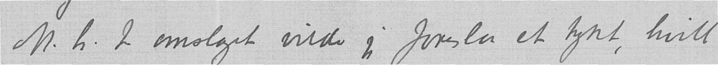M.h.t omslaget vilde jeg foreslå et tykt, hvitt
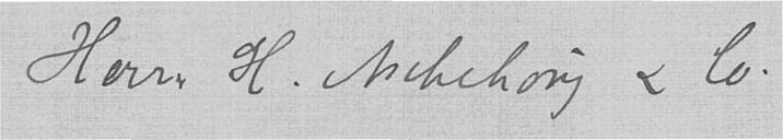Herrer H. Aschehoug & Co.
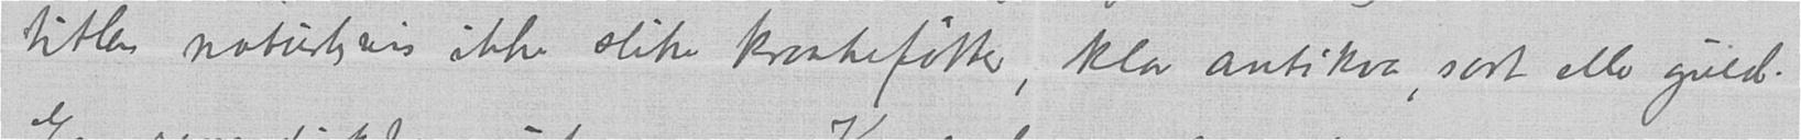titlen naturligvis ikke slike kraakeføtter, klar antikva, sort eller guld.
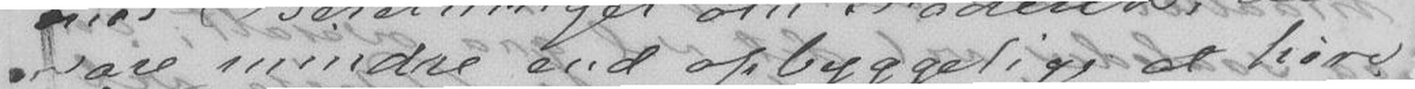vare mindre end opbyggelig at høre
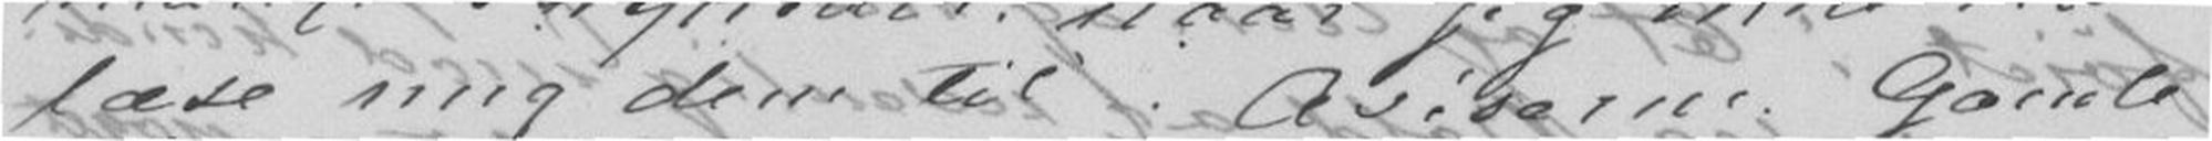læse mig dem til i Aviserne. Gamle
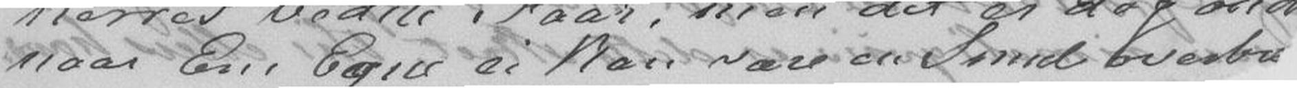naar Ens Egne ei kan være en Sund overbæ
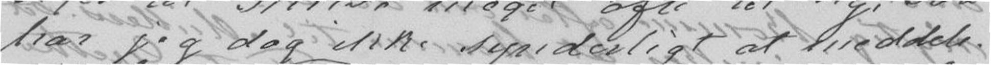har jeg dog ikke synderligt at meddele.
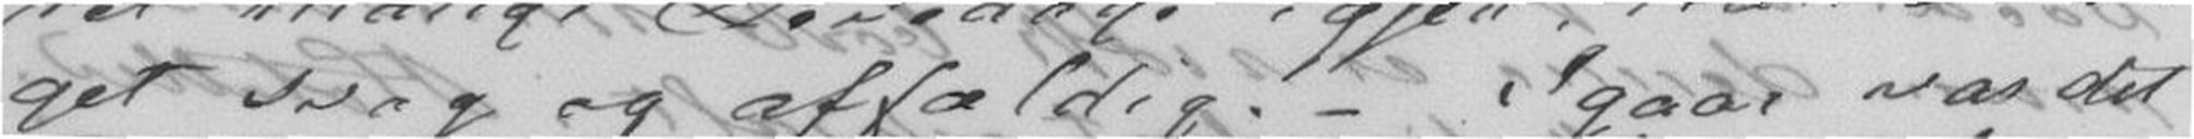get svag og affældig. - Igaar var det
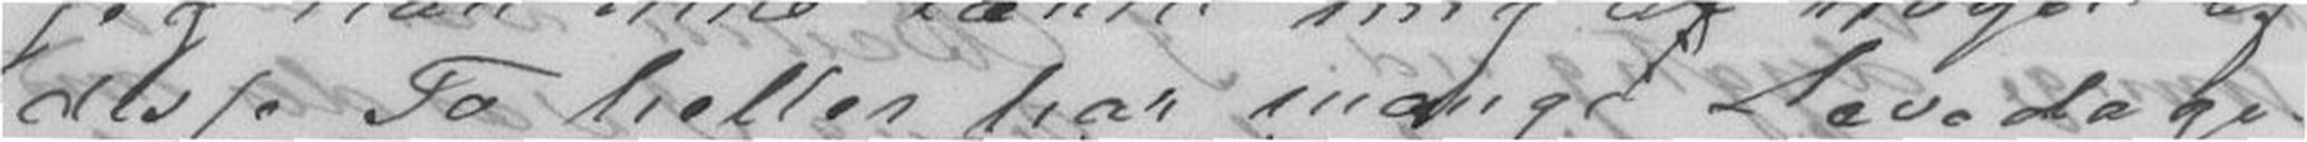disse To heller har mange Levedage.
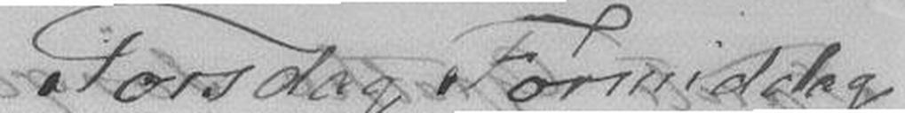Torsdag Formiddag
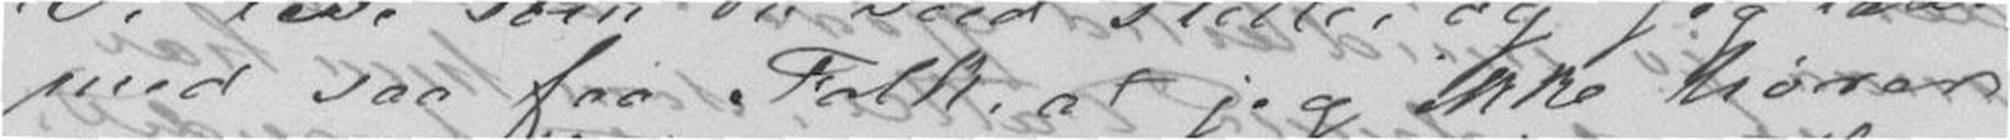med saa faa Folk, at jeg ikke hører
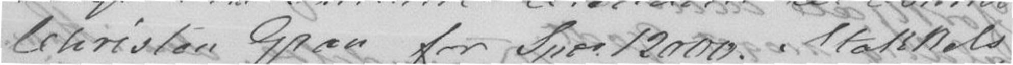Christen Gran for Spdo 12000. Stakkels
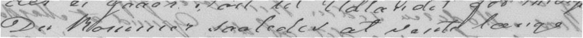Du kommer saaledes at vente længe
